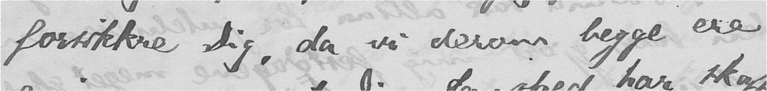forsikkre Dig, da vi derom begge ere
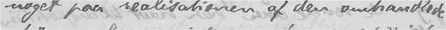noget paa realisationen af den omhandlede
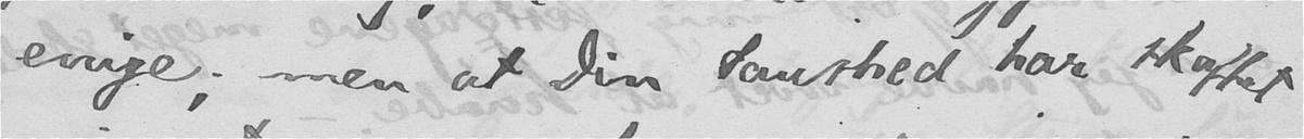enige; men at Din taushed har skaffet
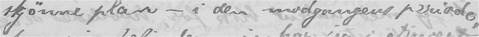skjønne plan – i den modgangens periode,
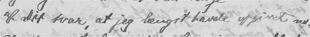af Dit svar, at jeg længst havde opgivet no-
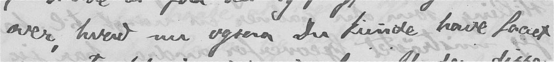over, hvad nu ogsaa Du kunde have faaet
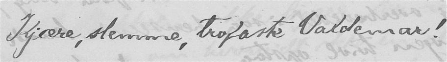Kjære, slemme, trofaste Valdemar!
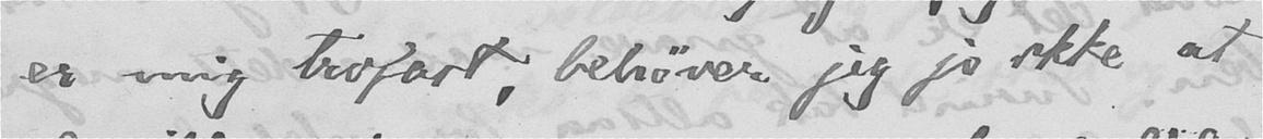er mig trofast, behøver jeg jo ikke at
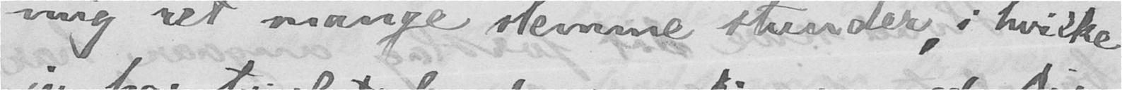mig ret mange slemme stunder, i hvilke
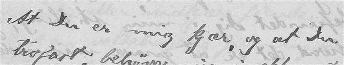At Du er mig kjær, og at Du
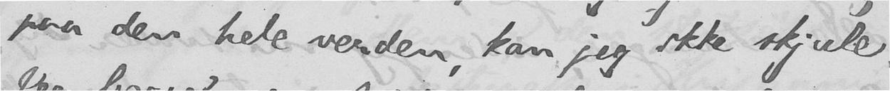paa den hele verden, kan jeg ikke skjule.
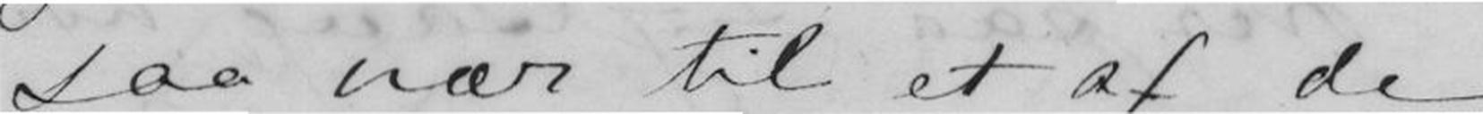saa nær til et at de
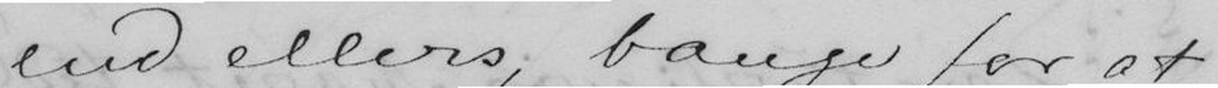end ellers, bange for at
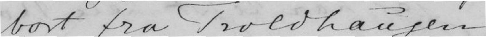bort fra Troldhaugen.
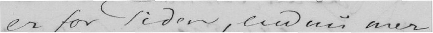er for Tiden, endnu mer
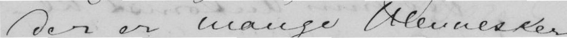der er mange Mennesker
